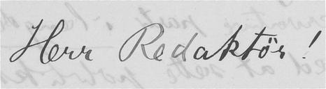Herr Redaktør.
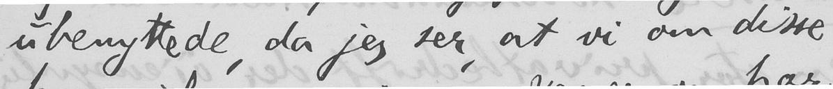ubenyttede, da jeg ser, at vi om disse
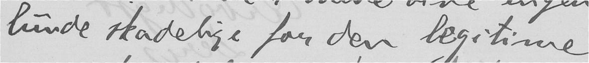lunde skadelige for den legitime
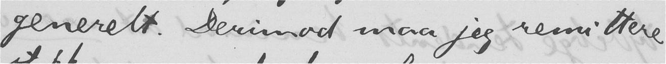generelt. Derimod maa jeg remittere
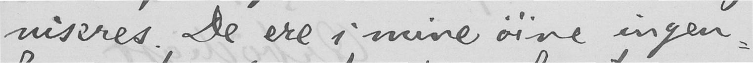niseres. De ere i mine øine ingen-
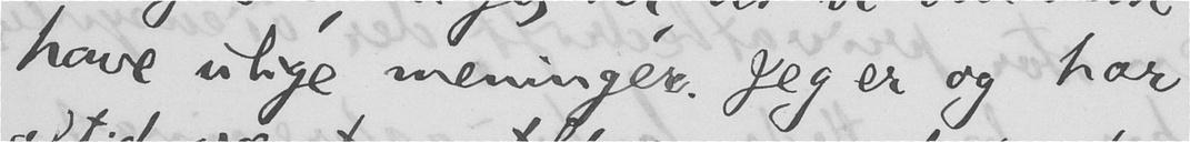have ulige meninger. Jeg er og har
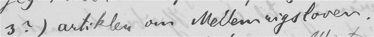3?) artikler om Mellemrigsloven.
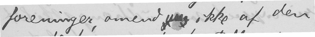foreninger, omend  ikke af den
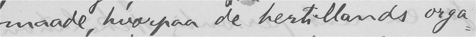maade, hvorpaa de hertillands orga-
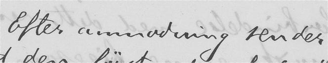Efter anmodning sender
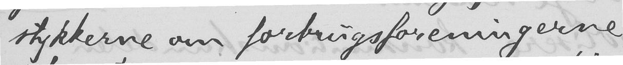stykkerne om forbrugsforeningerne
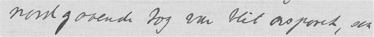nordgaaende tog var blit avsporet, saa
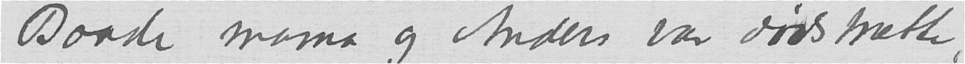Baade mama og Anders var dødstrætte,
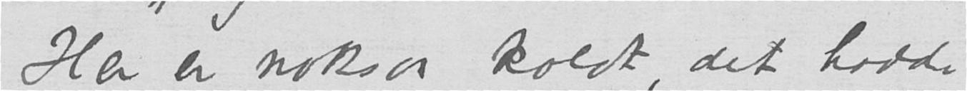Her er noksåa koldt, det hadde
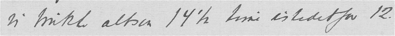vi brukte altsaa 14½ time istedetfor 12.
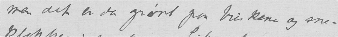men det er da grønt paa buskene og sne-
